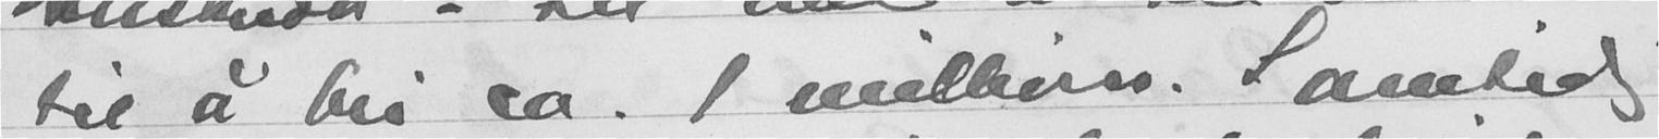til å bli ca. 1 millon. Samtidig
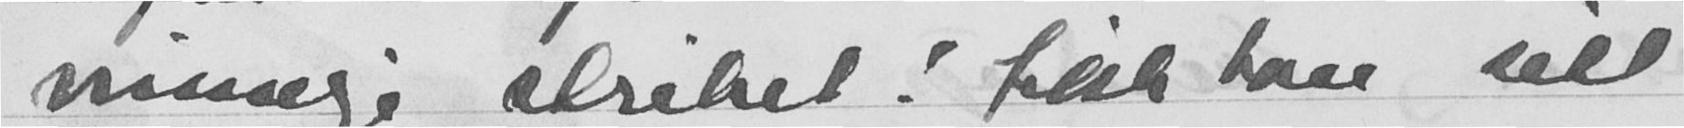vinnelige strihet! fikk han sitt
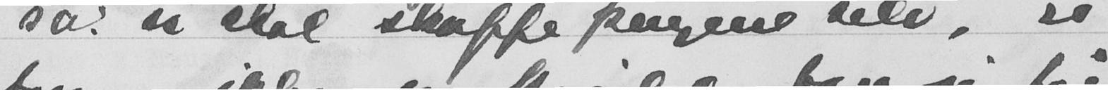sa: vi skal skaffe pengene selv, vi
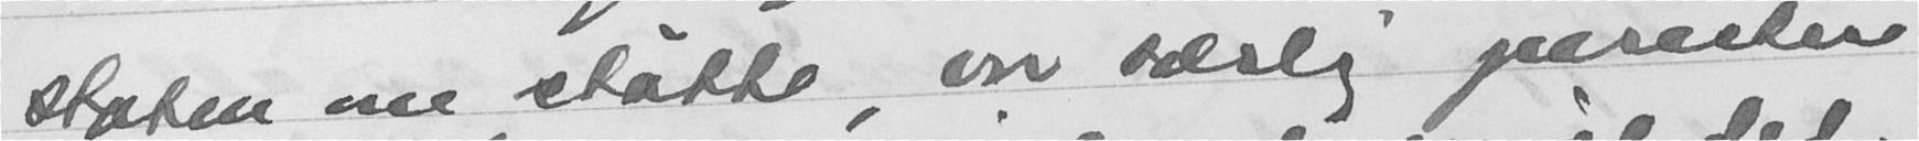staten om støtte, var særlig juseiter
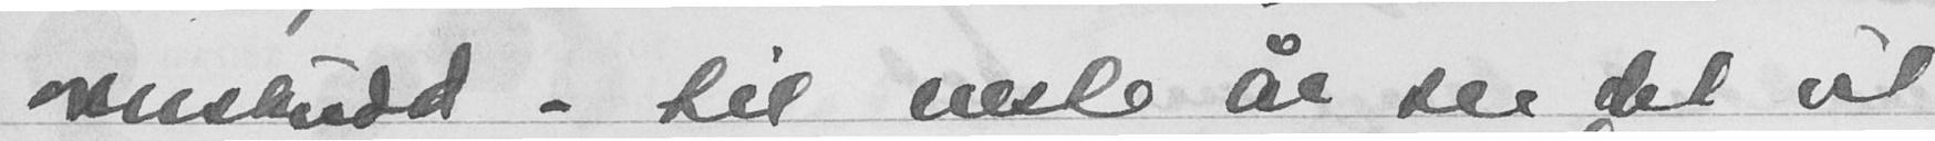overskudd - til neste år ser det ut
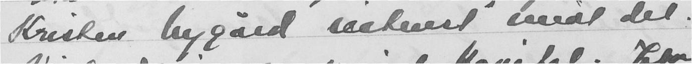Kristen Nygård intenst imot det.
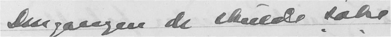Dengangen de skulde søke
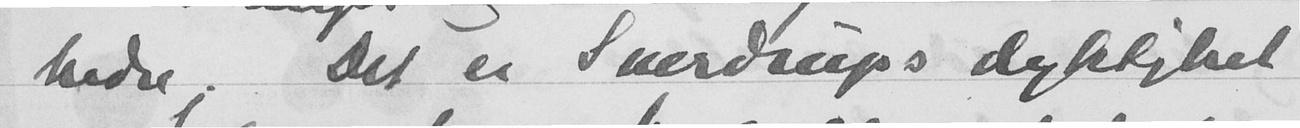bedre. Det er Sverdrups dyktighet
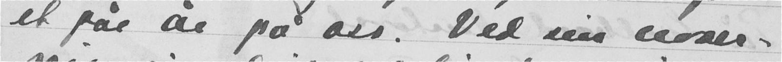et par år på oss. Ved sin uover-
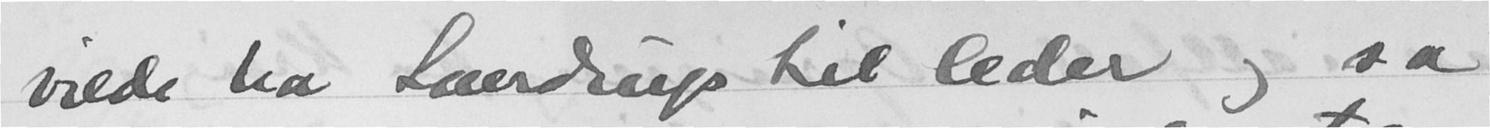vilde ha Sverdrups til leder og sa
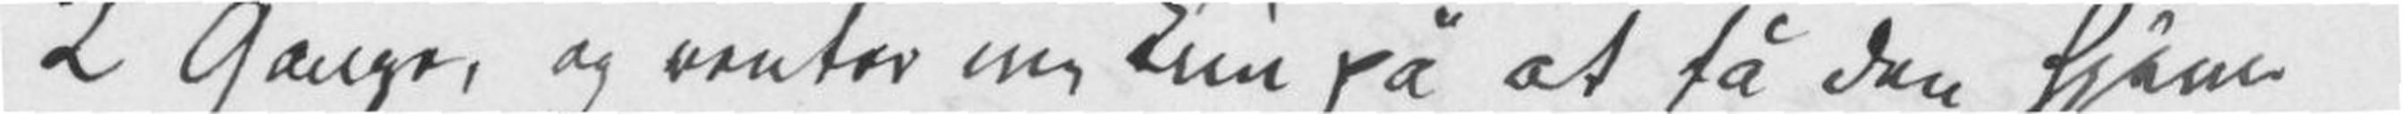2 Gange, og venter nu kun på at få den hjem
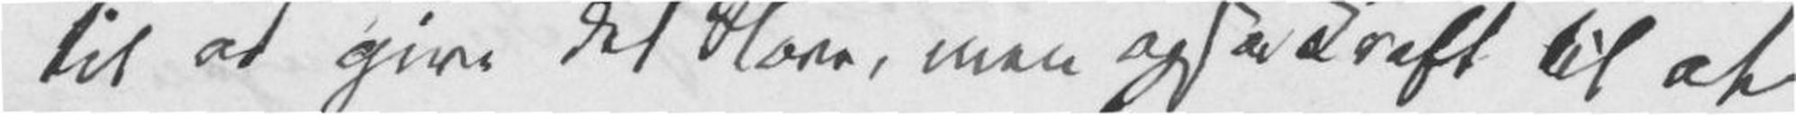til at give det Store, men også Kraft til at
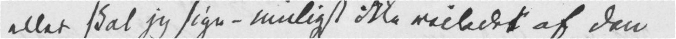eller skal jeg sige – muligst ikke veiledet af den
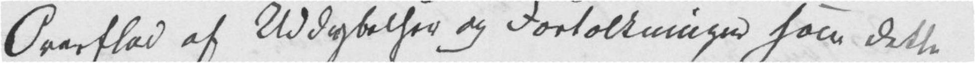Overflod af Uddybelser og Fortolkninger som dette
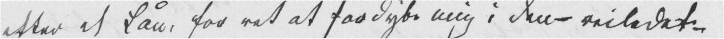efter et Lån, for ret at fordybe mig i den – veiledet –
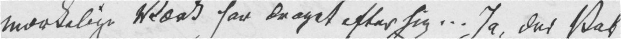mærkelige Værk har draget efter sig ... Ja, der skal
